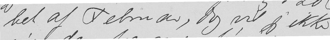bet af Februar, dog vil jeg ikke
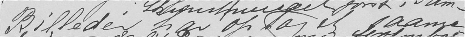Billeder har optaget saame-
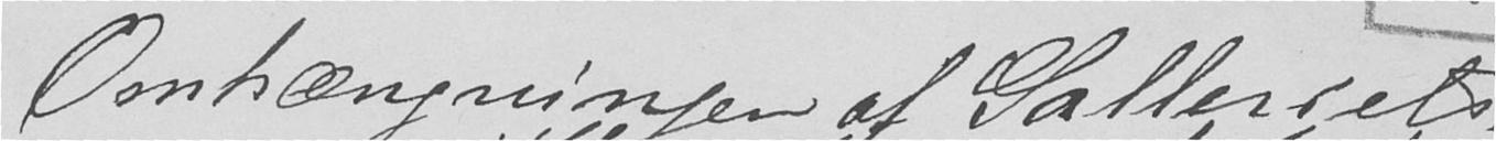Omhængningen af Galleriets
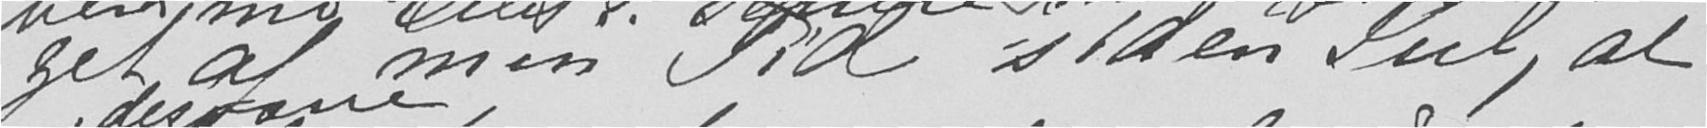get af min Tid siden Jul, at
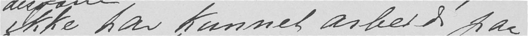ikke har kunnet arbeide paa
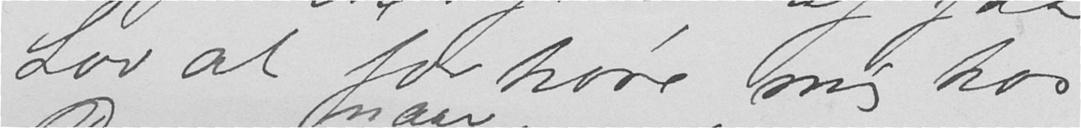Lov at forhøre mig hos
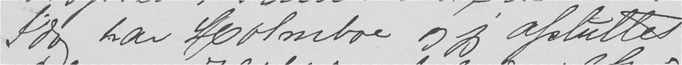Idag har Holmboe og jeg afsluttet
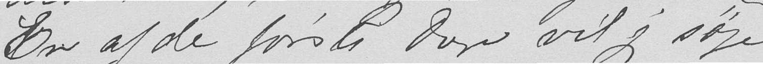En af de første dage vil jeg søge
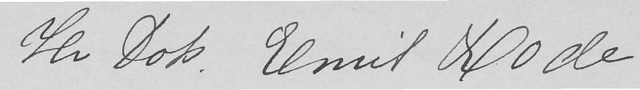Hr Dok. Emil Rode.
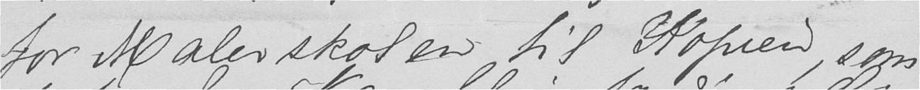for Malerskolen til Kopien, som
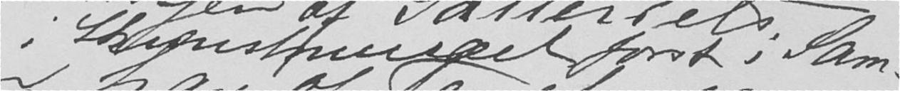i Kunstmusæet først i Sam-
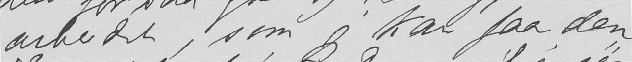arbeidet, som jeg kan faa den.
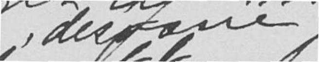desværre
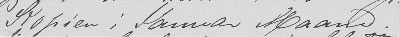Kopien i Januar Maaned.
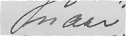naar
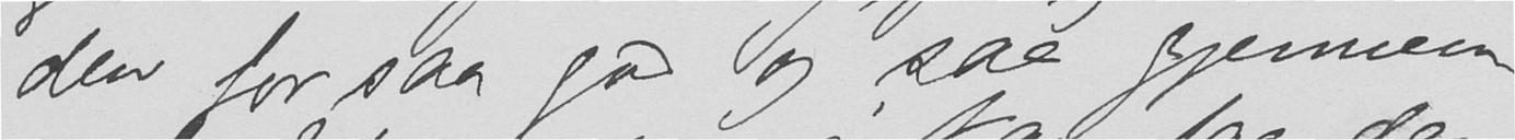den for saa god og saa gjennem-
